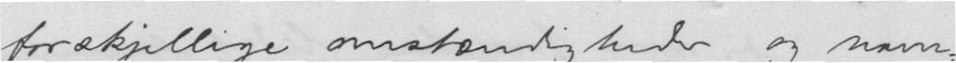forskjellige omstendigheder og næm-
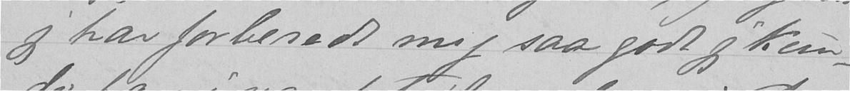jeg har forberedt mig saa godt jeg kun-
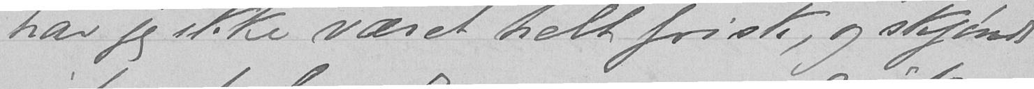har jeg ikke været helt frisk, og skjøndt
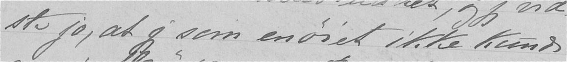ste jo, at jeg som enoiet ikke kunde
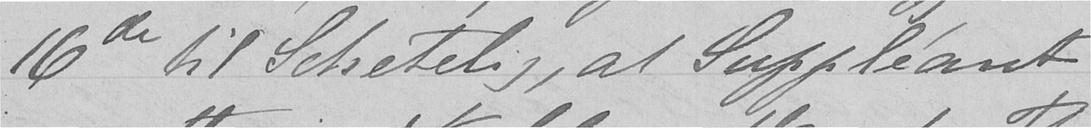16de til Schetelig, at Suppleant
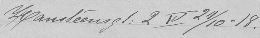Hansteensgt. 2 IV 24/10-18.
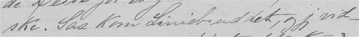ske. Saa kom Liniebruddet, og jeg vid-
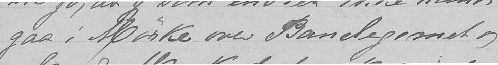gaa i Mørke over Banelegemet og
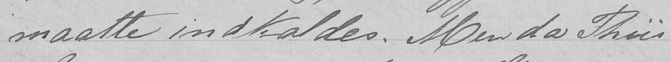maatte indkaldes. Men da Thiis
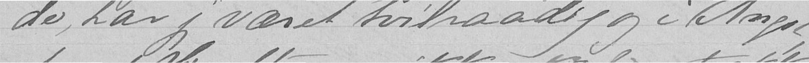de, har jeg været tvilraadig og i Angst
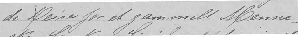de Reise for et gammelt Menne-
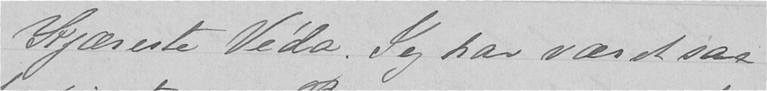Kjæreste Veda. Jeg har været saa
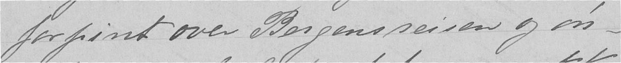forpint over Bergensreisen og øn-
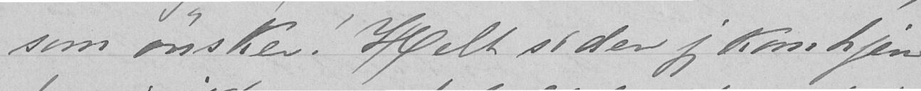som ønsker. Helt siden jeg kom hjem
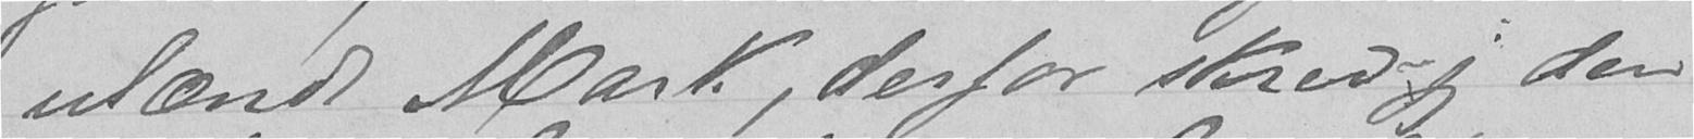ulændt Mark, derfor skrev jeg den
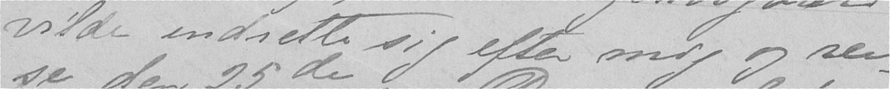vilde indrette sig efter mig og rei-
